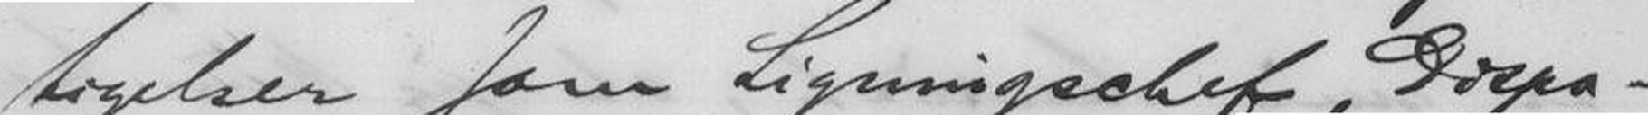tigelser som Ligningschef, Dispa-
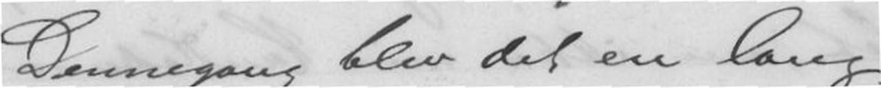Dennegang blev det en lang
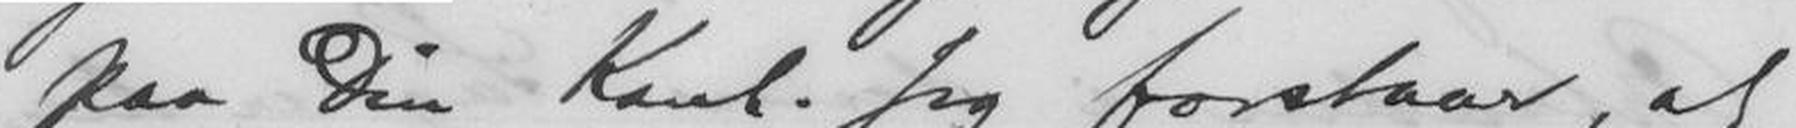paa Din Kant. Jeg forstaar, at
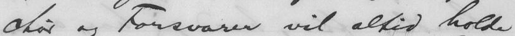chør og Forsvarer vil altid holde
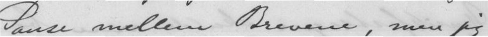Pause mellem Brevene, men jeg
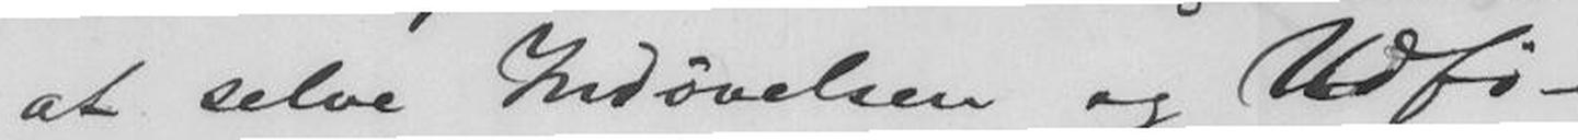at selve Indøvelsen og Udfø-
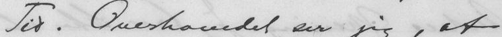Tid. Overhovedet ser jeg, at
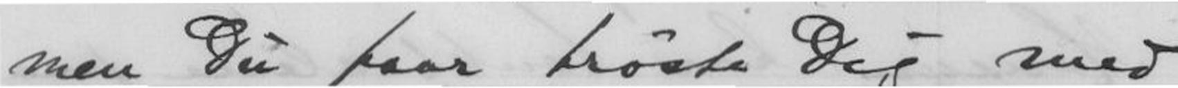men Du faar trøste Dig med
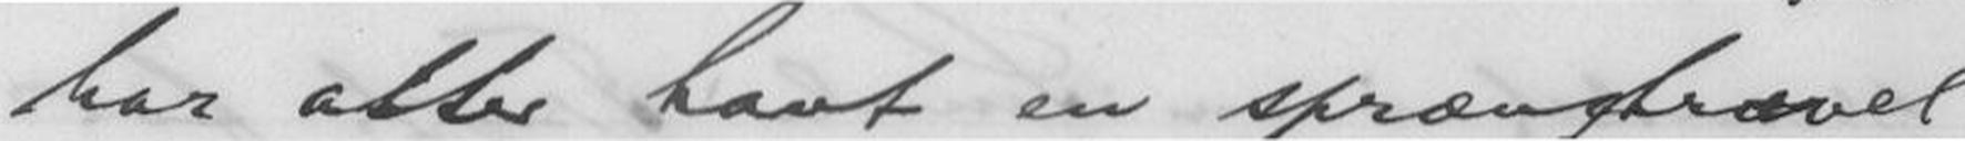har atter havt en sprængtravel
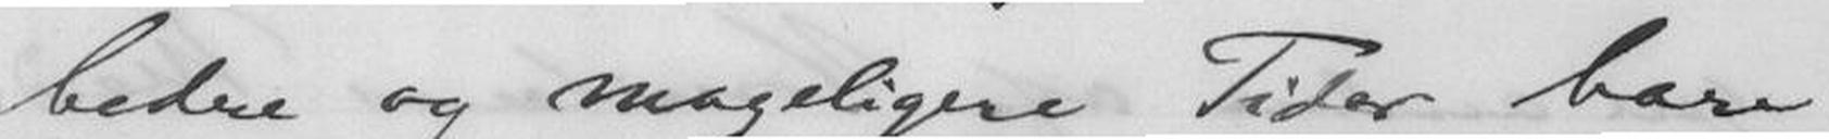bedre og mageligere Tider bare
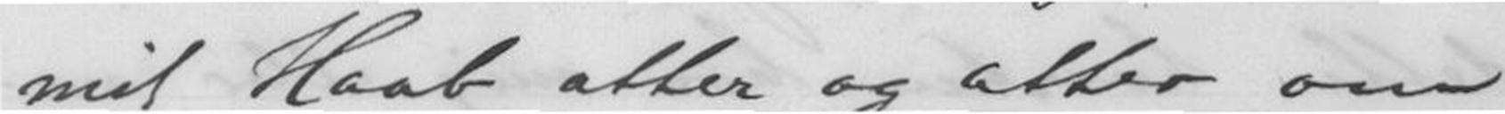mit Haab atter og atter om
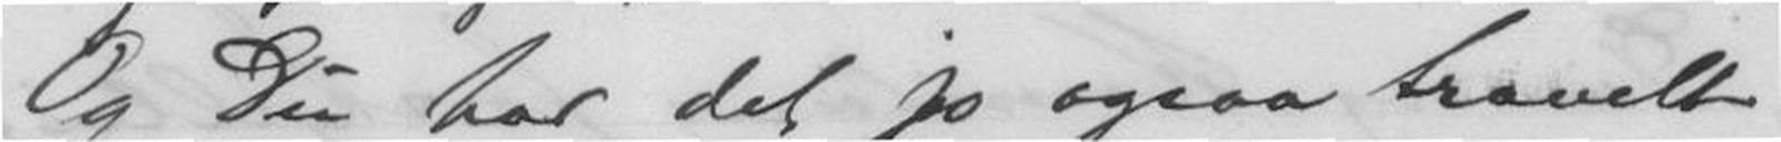Og du har det jo ogsaa travelt
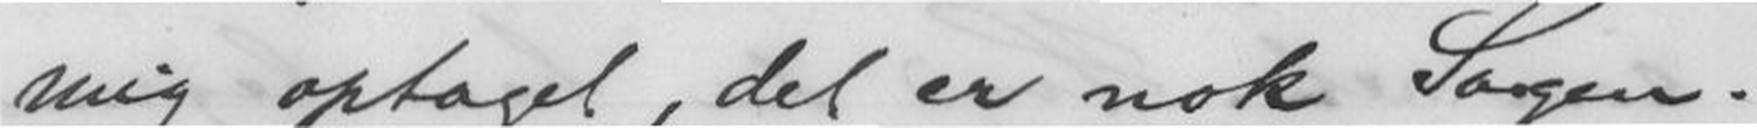mig optaget, det er nok Sagen.
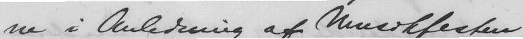ne i Anledning af Musikfesten,
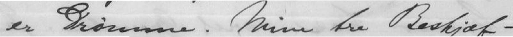er Grønne. Mine tre Beskjæf-
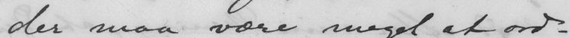der maa være meget at ord-
